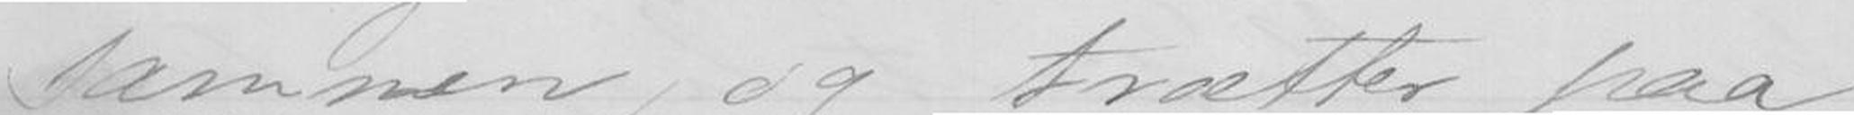sammen, og trætter paa
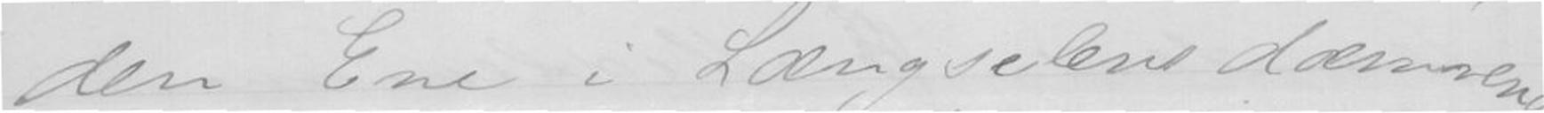den Ene i Længselens dærverende
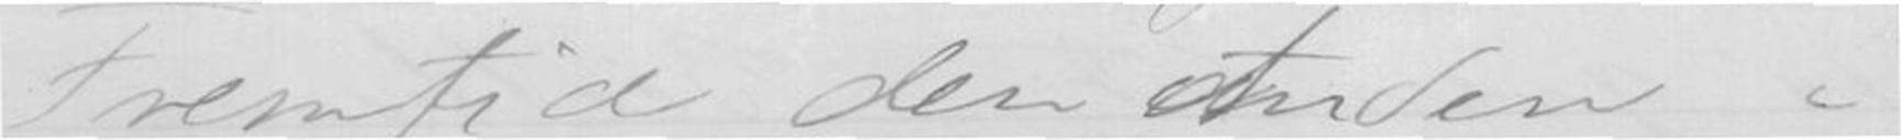Fremtid, der Anden i
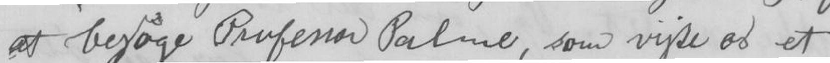at besøge Professor Palme, som viste os et
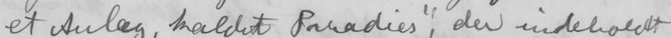et Anlæg, kaldet Paradies, der indeholdt
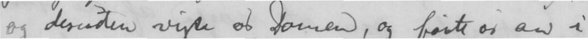og desuden viste os Domen, og førte os an i
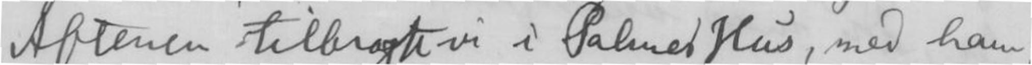Aftenen tilbragte vi i Palmes Hus, med ham,
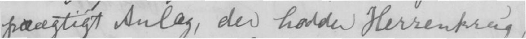prægtigt Anlæg, der hadde Herrenkrug,
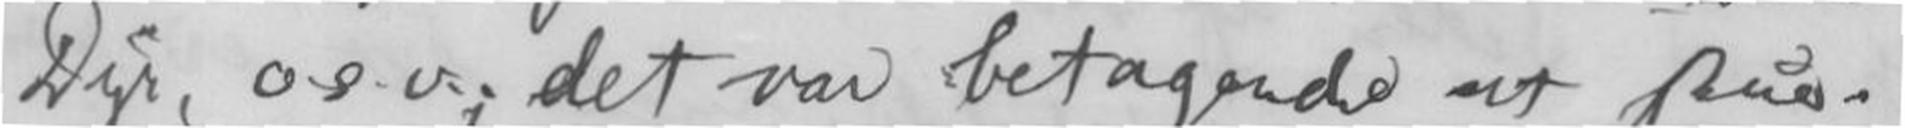Dyr, o.s.v.; det var betagende at skue.
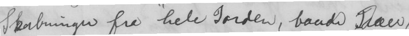Skabninger fra hele Jorden, Trær,
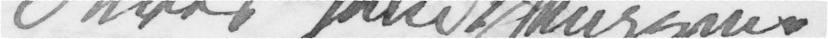Deres sandt hengivne
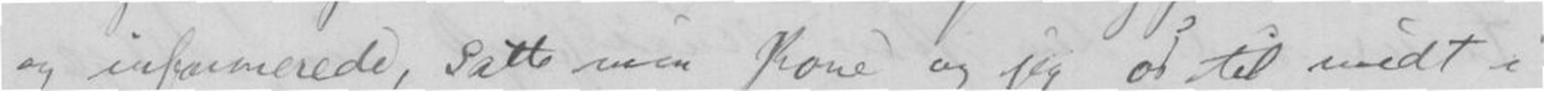og informerede, satte min Kone og jeg os til midt i
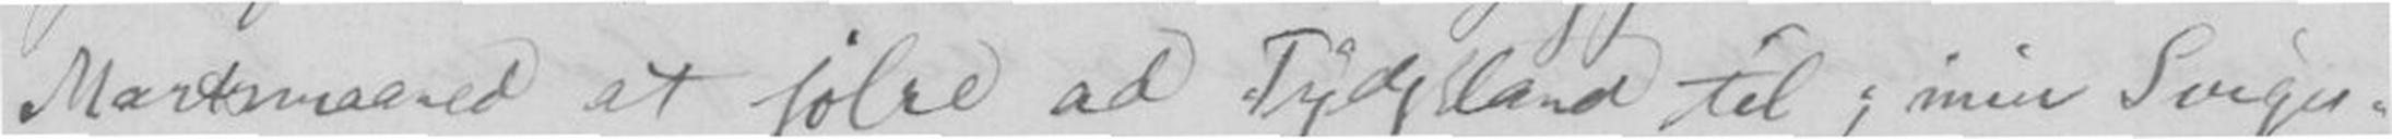Martsmaaned at sølre ad Tydskland til; min Sviger-
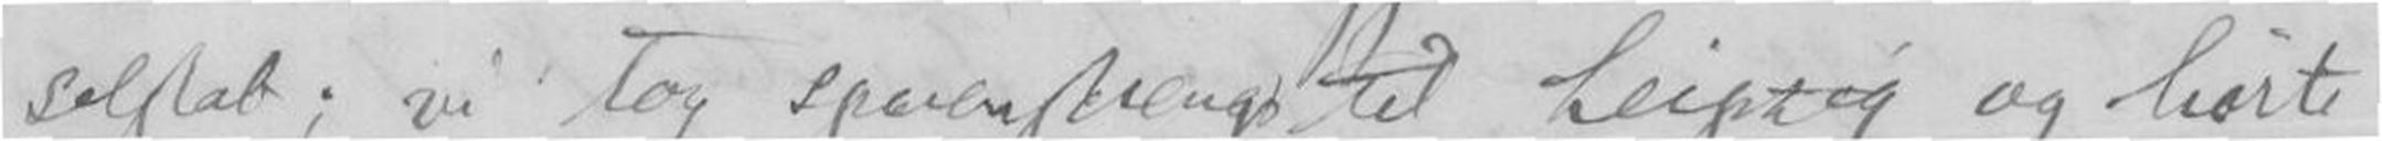selskab; vi tog sporenstrengs til Leipzig og hørte
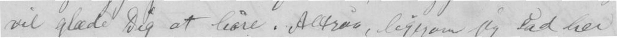vil glæde Dig at høre. Altsaa, ligesom jeg sad her
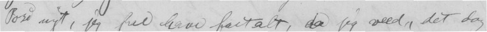Pose nyt, jeg skal have fortalt, da jeg veed, det dog
